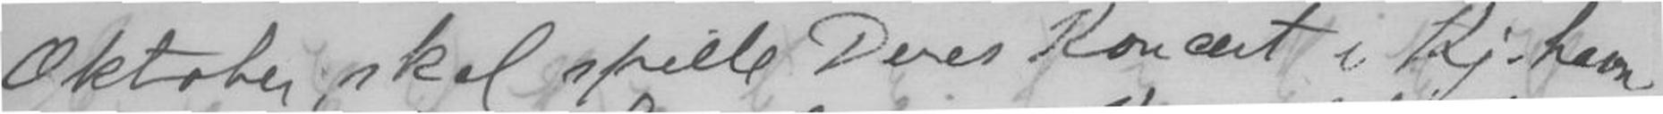Oktober, skal spille Deres Koncert i Kj.havn
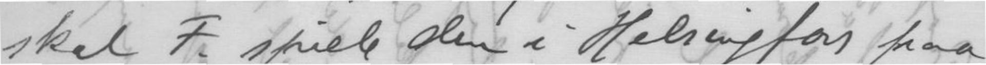skal F. spille den i Helsingfors paa
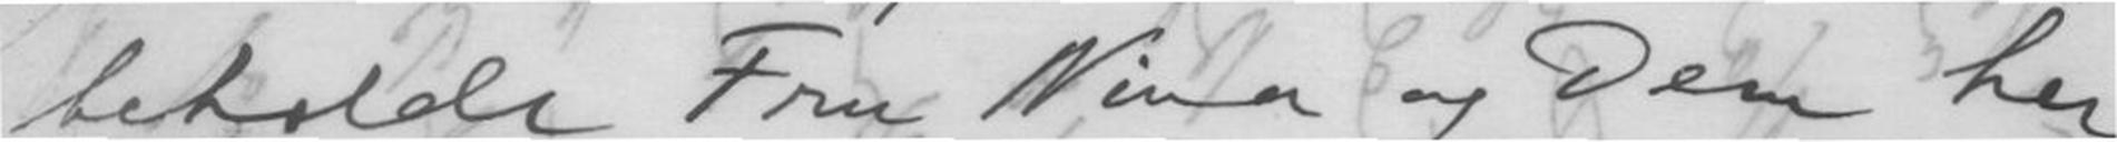beholde Fru Nina og Dem her
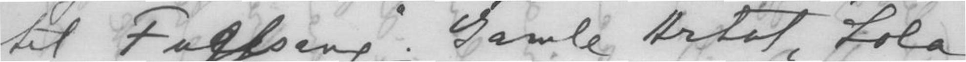til Fuglsang. Gamle Artót, Lola
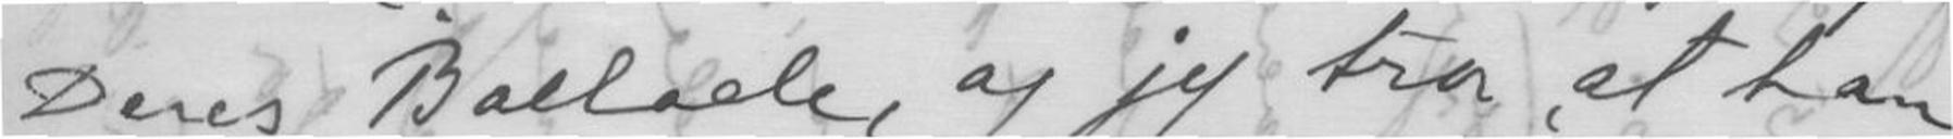Deres Ballade, og jeg tror, at han
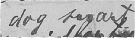dog snart
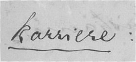karriere:
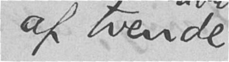af tvende
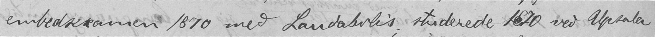embedsexamen 1870 med Laudabilis; studerede 1870 ved Upsala
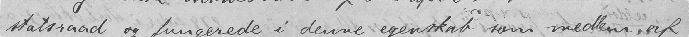statsraad og fungerede i denne egenskab som medlem af
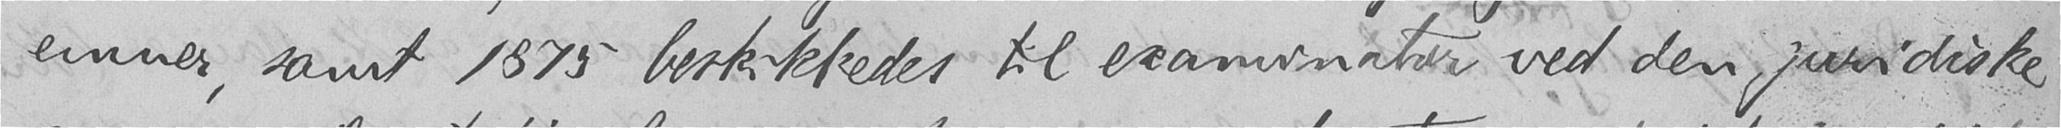emner, samt 1875 beskikkedes til examinator ved den juridiske
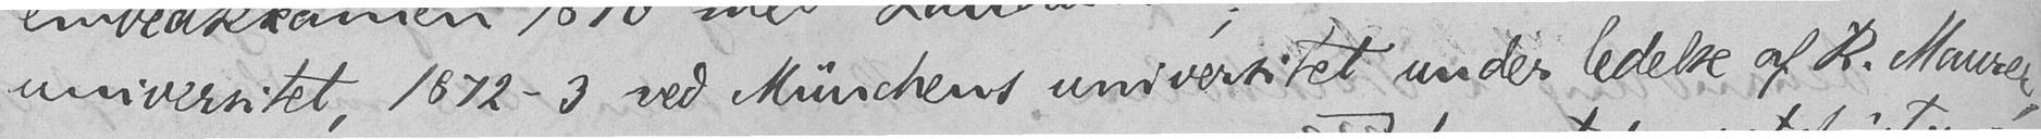universitet, 1872-3 ved Münchens universitet under ledelse af K. Maurer,
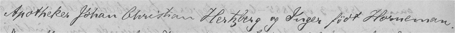Apotheker Johan Christian Hertzberg og Inger født Horneman.
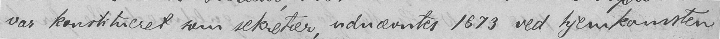var konstitueret som sekretær, udnævntes 1873 ved hjemkomsten
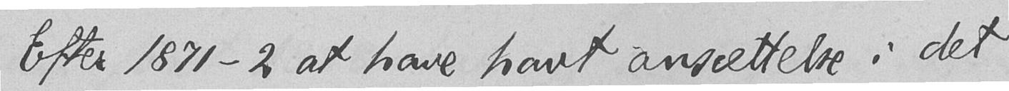Efter 1871-2 at have havt ansættelse i det
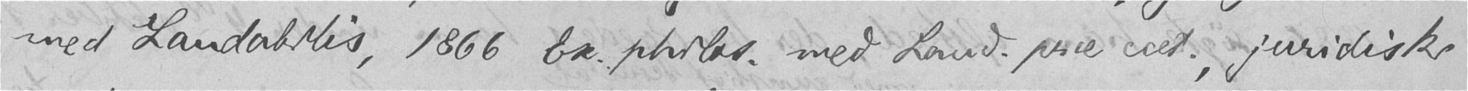med Laudabilis, 1866 Ex. philos. med Laud. præ ccet., juridisk
